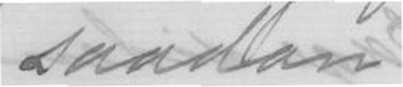saadan
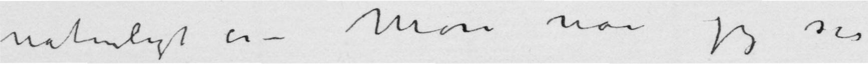naturligt er – Mon når jeg ser
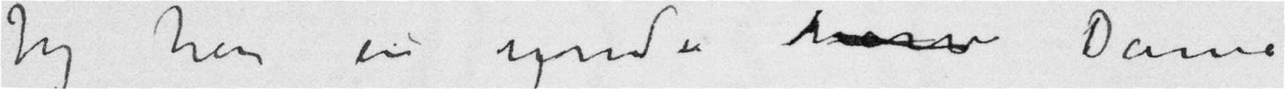Jeg har en yndig Dame
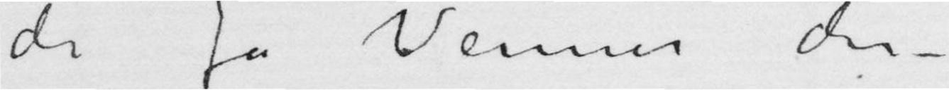de få Venner der –
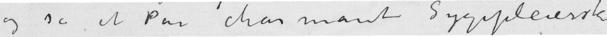og så et par charmante Sygepleiersker
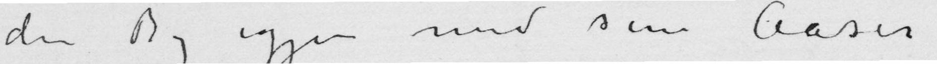den By igjen med sine Aaser
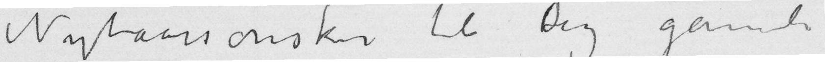Nytaarsønsker til Dig gamle
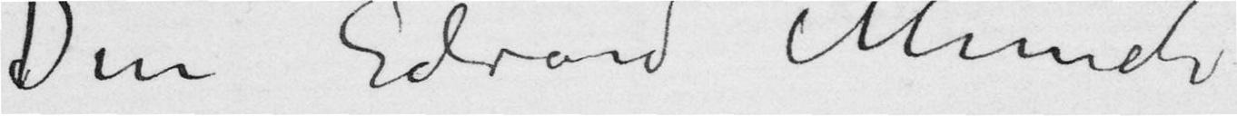Din Edvard Munch
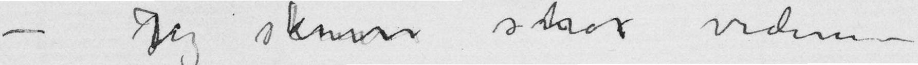– Jeg skriver strax videre
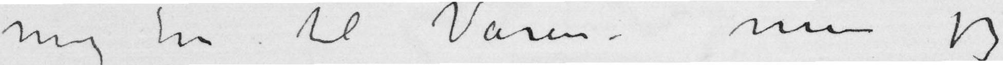mig her til Våren – men jeg
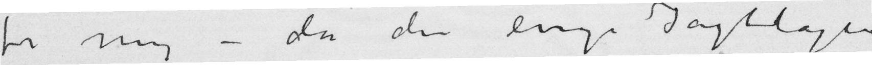for mig – da den evige Iagtagelse
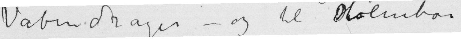Våbendrager – og til Holmboe
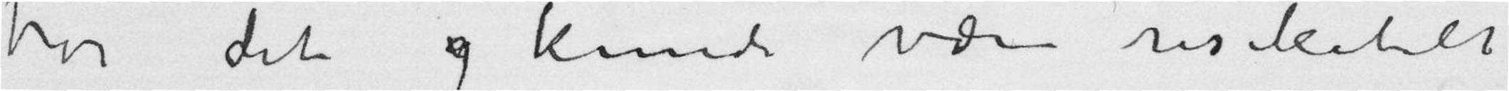tror det g kunde være resikabelt
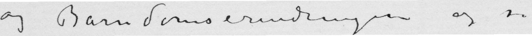og Barndomserindringer og så
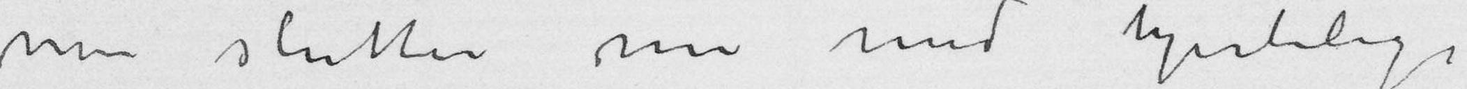men slutter nu medhjertelige
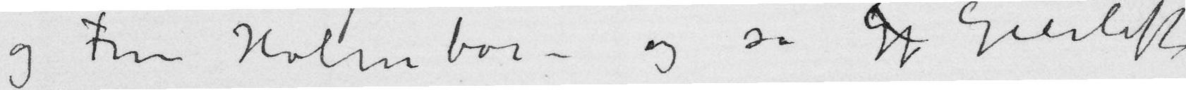og Fru Holmboe – og så gj Gierløff
